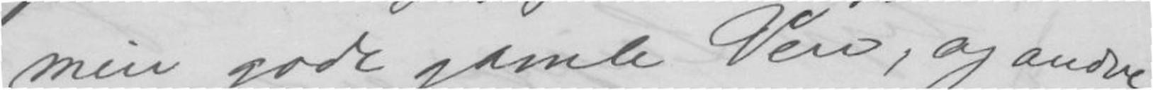min gode gamle Ven, og andre
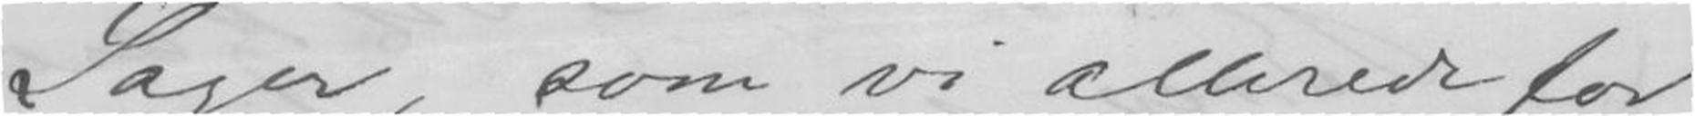Sager, som vi allerede for
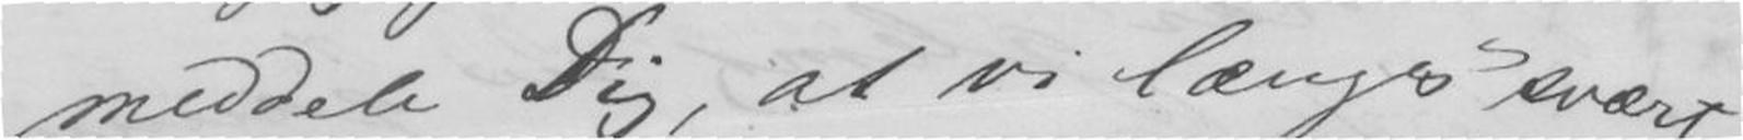meddele Dig, at vi længes svært
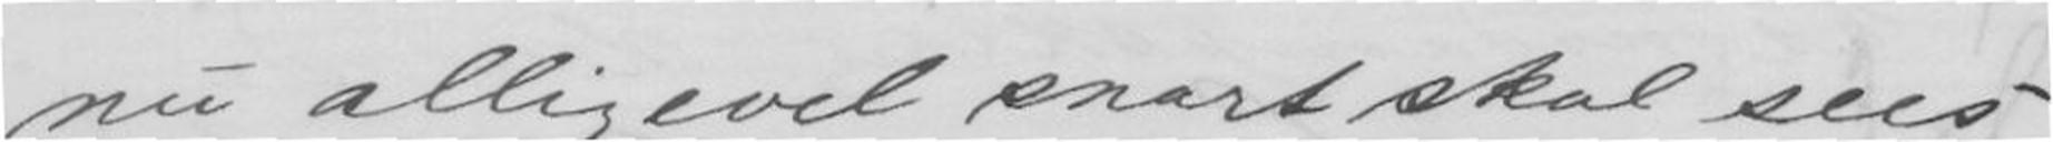nu alligevel snart skal sées
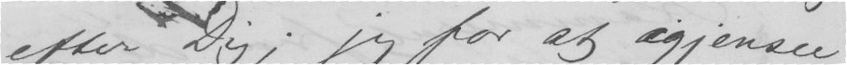efter Dig; jeg for at igjensee
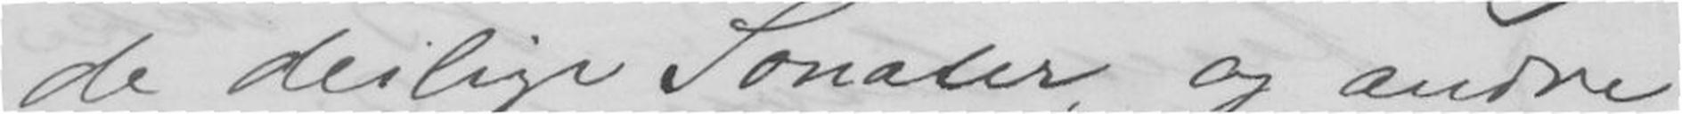de deilige Sonater og andre
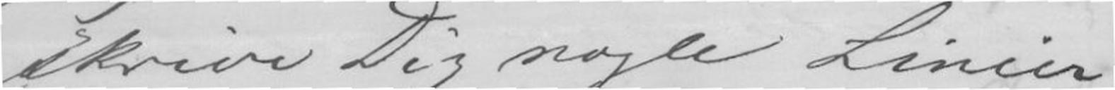skrive Dig nogle Linier
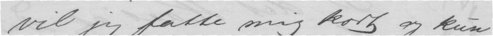vil jeg fatte mig kort og kun
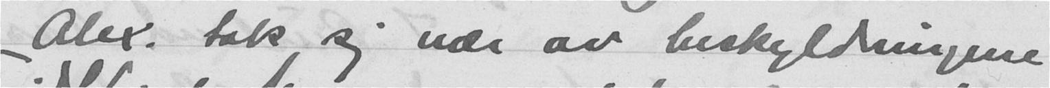Alex. tok sig nær av beskyldningene
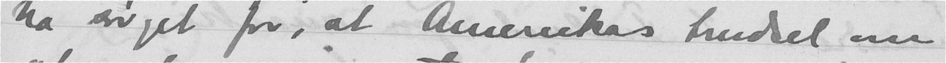ha sørget for, at Amerikas trudsel om
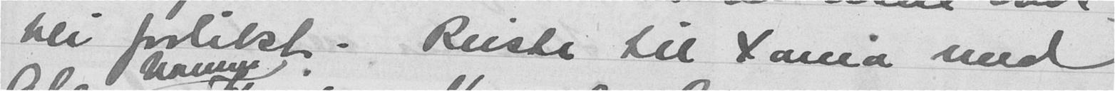bli forlikt. Reiste til Xania med
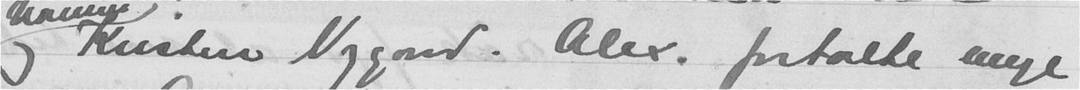og Kristen Nygaard. Alex. fortalte mye
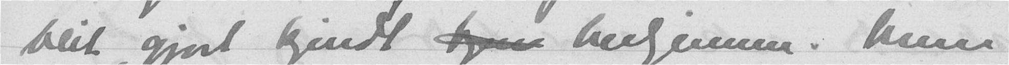blit gjort kjendt hjem herhjemme. Men
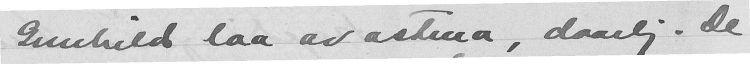Gunhild laa av astma, daarlig. De
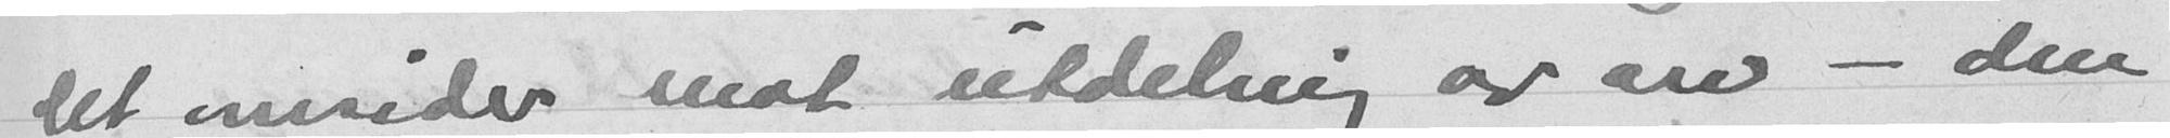det omsider mot utdeling av arv - den
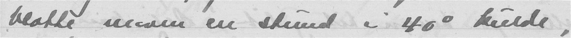blotte maven en stund i 40° kulde,
